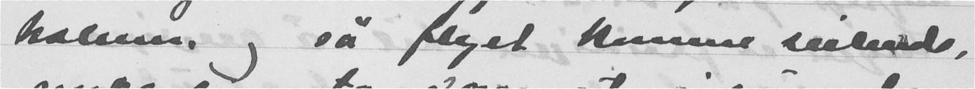holmen, og så flyet komme seilende,
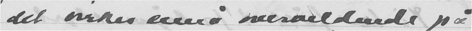det virker ennå overveldende på
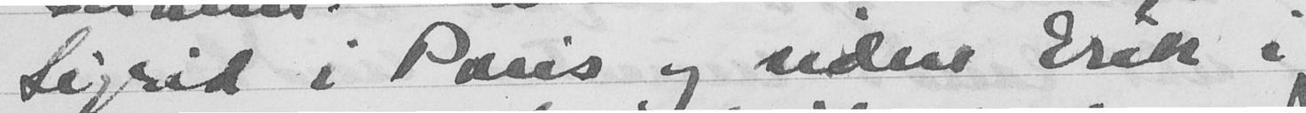Sigrid i Paris og siden Erik i
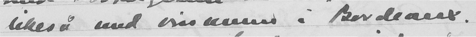likeså med vinmenn i Bordeaux.
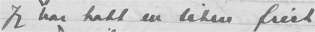Jeg har hatt en liten trist
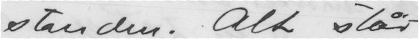tanden. Alt står
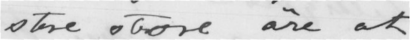store store äre at
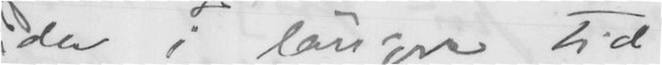da i längre Tid
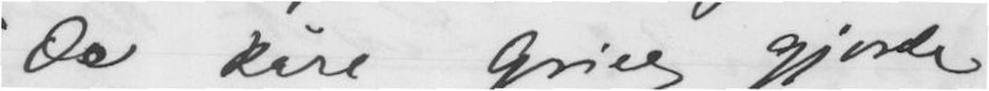De käre Grieg gjorde
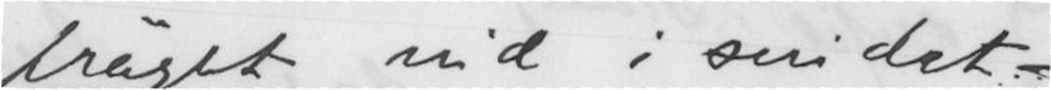präget ind i sindet. -
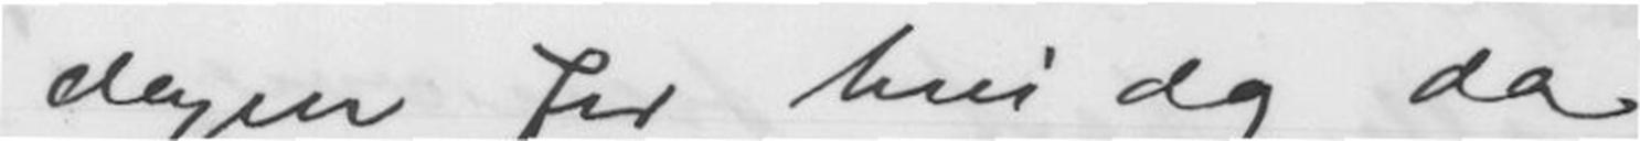dagen for hin dag da
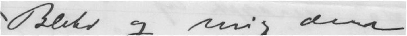Blehr og mig den
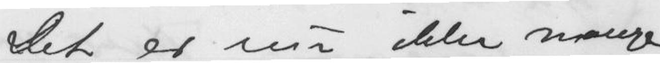Det er nu ikke mange
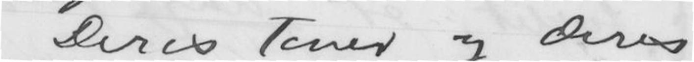Deres Toner og Deres
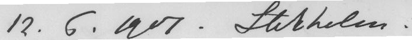12.6.1901.Stokholm.
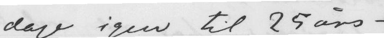dage igjen til 25 års-
